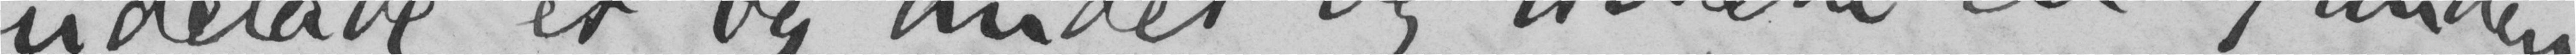udelade et og andet og tilsætte en og anden
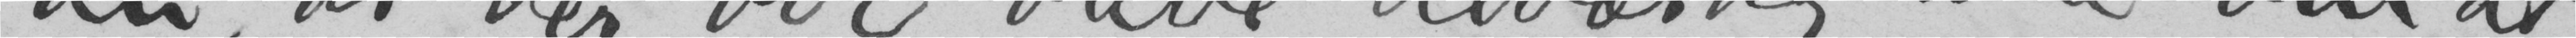nu, at der bør blive alvorlig tale om at
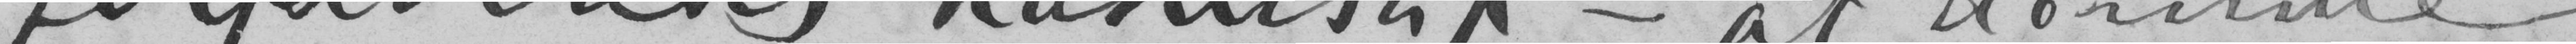forfatterens kasuistik - at dømme
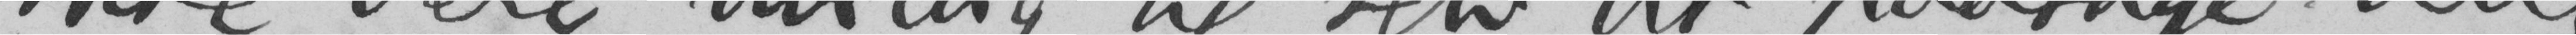ikke være uvillig til selv at paatage mig
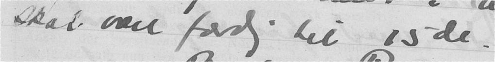skal være ferdig til 15de.
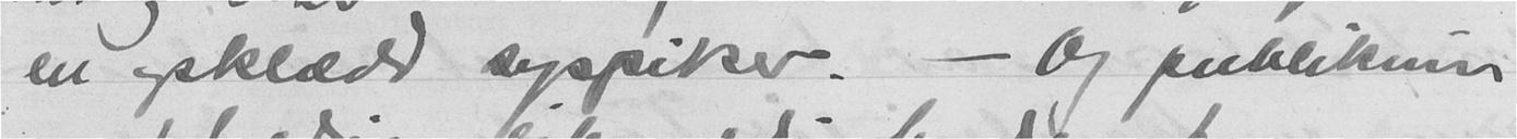en opklædt syspiker. - Og publikum
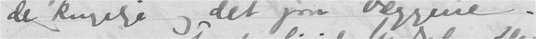de kongelige og det paa væggene.
